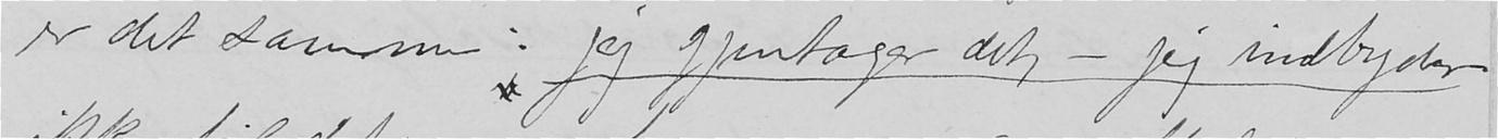er det samme: jeg gjentager det, - jeg indbyder
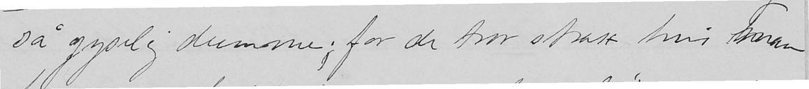så gyselig dumme; for de tror strax hvis man
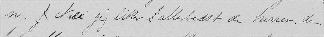ne. J Nei jeg liker allerbedst de herrer, der
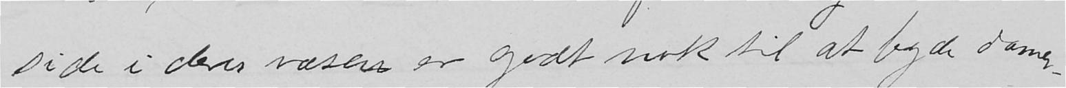side i deris væsen er godt nok til at byde damer-
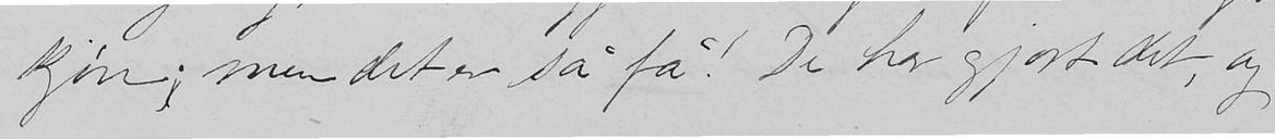kjøn; men det er så få! De har gjort det, og
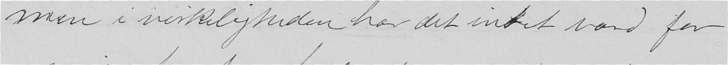men i virkeligheden har det intet værd for
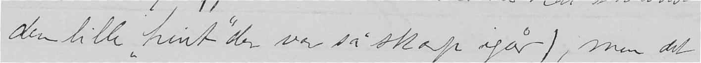den lille "hint" der var så skarp igar), men det
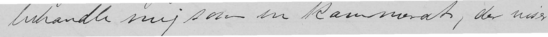behandler mig som en kammerat, der viser
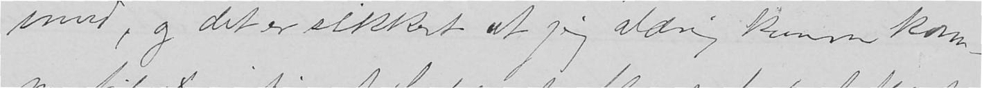imod, og det er sikkert at jeg aldrig kunne kom-
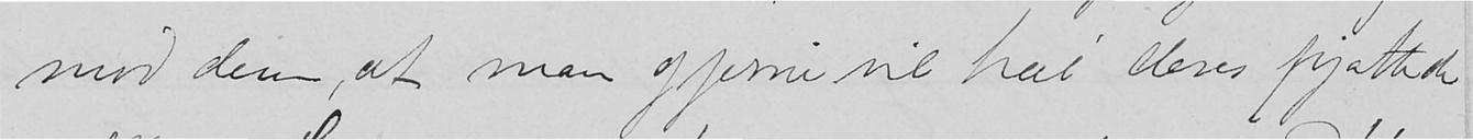mod dem, at man gjerne vil hae' deres pjattede
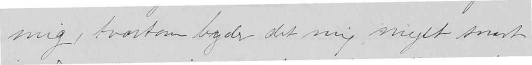mig, tværtom byder det mig meget svært
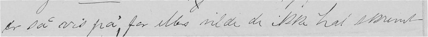er så vis på; for ellers vilde de ikke hae' skrevet
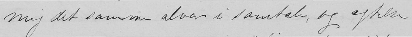mig det samme alvor i samtale, og agtelse
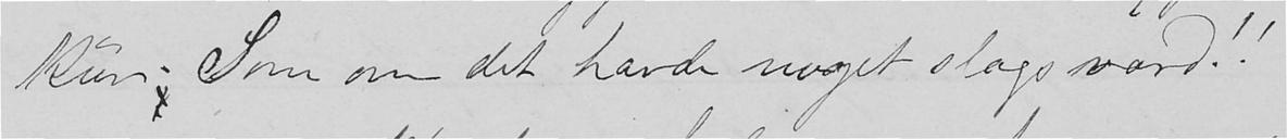kur. Som om det havde noget slags værd!!
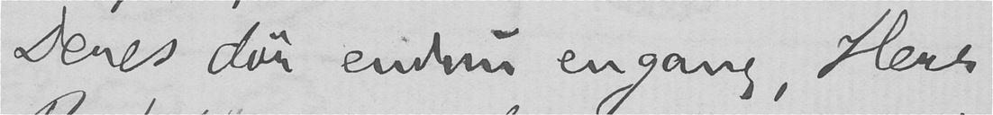Deres dør endnu engang, Herr
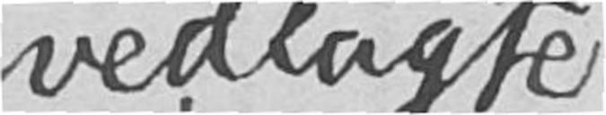vedlagte
